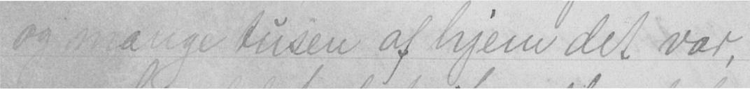og mange tusen af hjem det var,
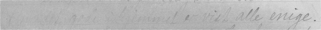det gode i hjemmet er vist alle enige.
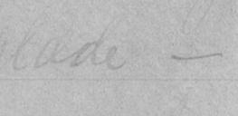glæde -
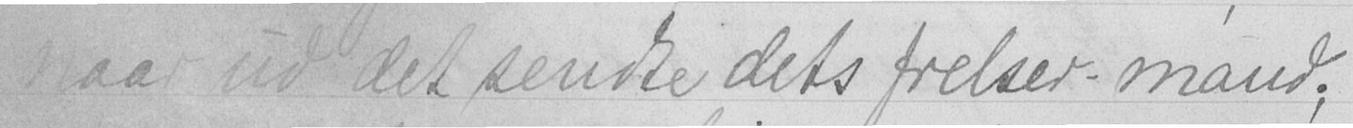naar ud det sendte dets frelser-mand;
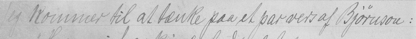Jeg kommer til at tænke paa et par vers af Bjørnson:
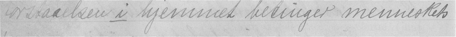forstaaelsen i hjemmet betinger menneskets
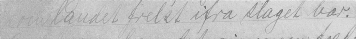som landet frelst ifra slaget bar.
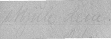skjule dem!
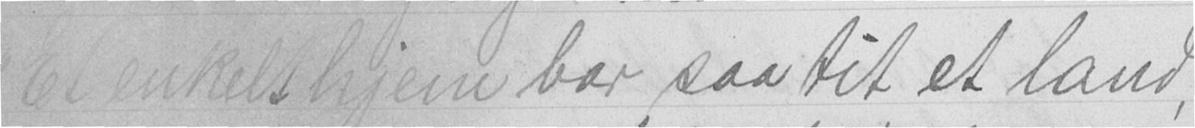Et enkelt hjem bar saa tit et land,
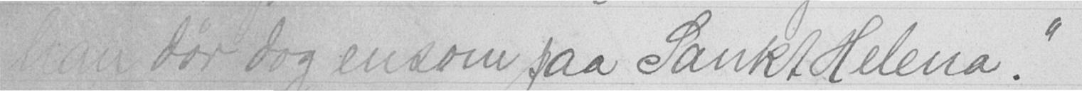han dør dog ensom paa Sankt Helena."
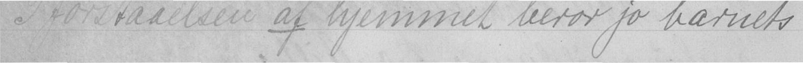I forstaaelsen af hjemmet beror jo barnets
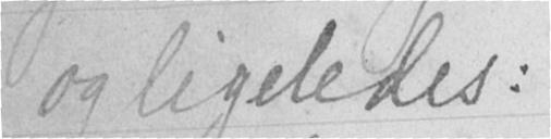og ligeledes:
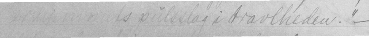er hjemmets pulsslag i travelheden." -
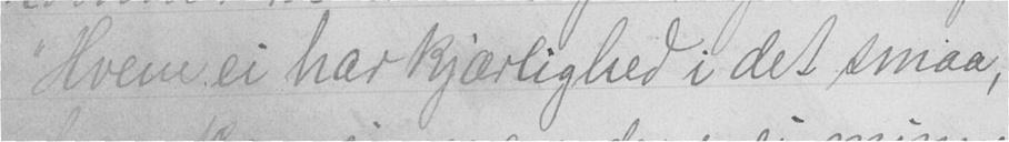"Hvem ei har kjærlighed i det smaa,
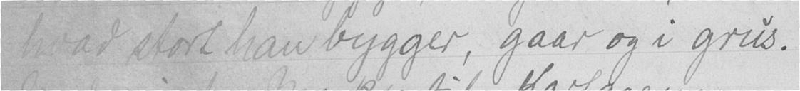hvad stort han bygger, gaar og i grus.
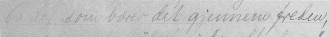Da det, som bærer det gjennem freden,
